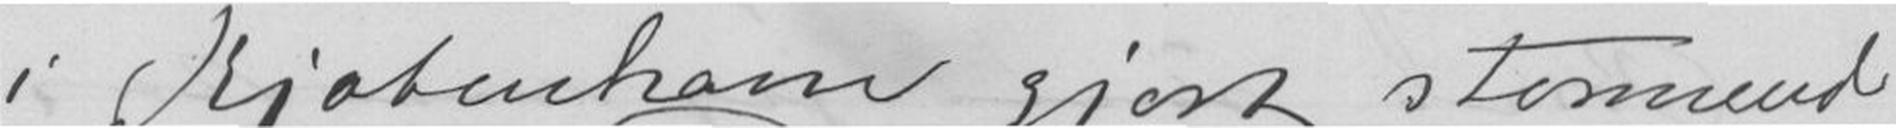i Kjøbenhavn gjort stormende
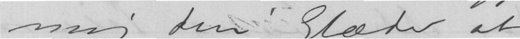mig den Glæde at
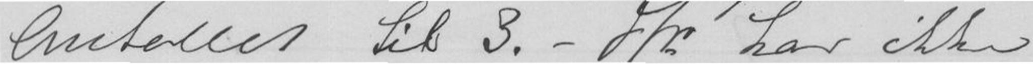Antallet til 3. - Dfr. har ikke
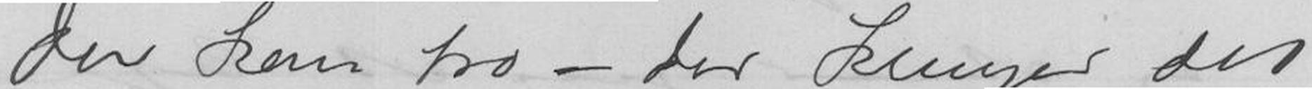Du kan tro - der klinger det
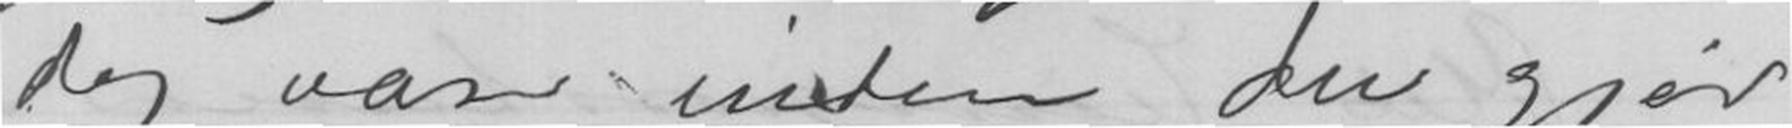dog være inden du gjør
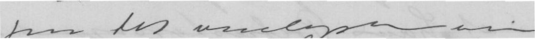paa det venligste vil
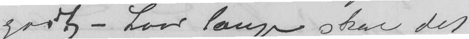godt - hvor længe skal det
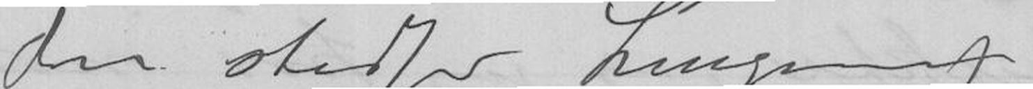Din stedse hengivne
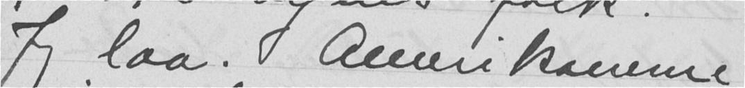Jeg laa. Amerikanerne
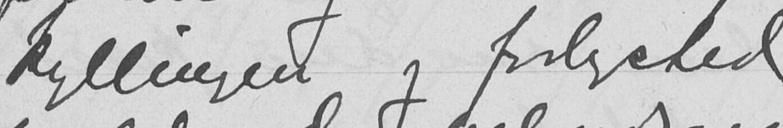kyllingen og forlysted
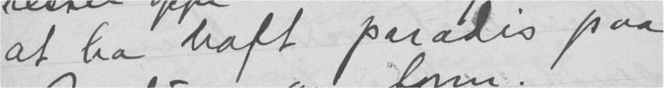at ha haft paradis paa
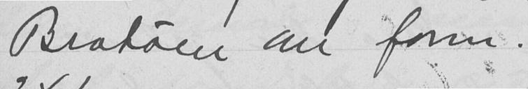Bratøen om form.
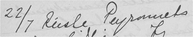22/7 Reiste Peyronnets
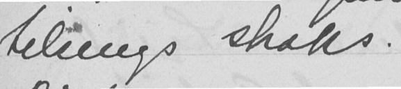tilsengs straks.
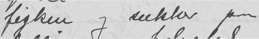fisken og sukker paa
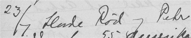23/7 Havde Rød og Peter
